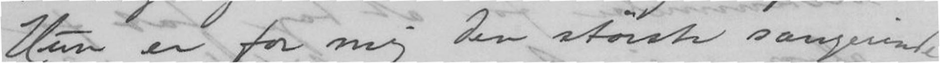Hun er for mig den største sangerinde
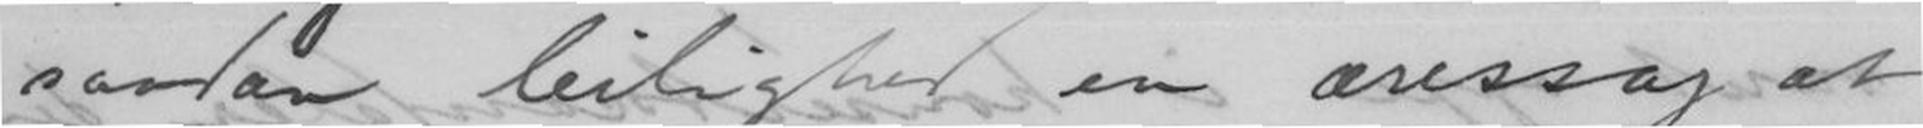saadan leilighed en æressag
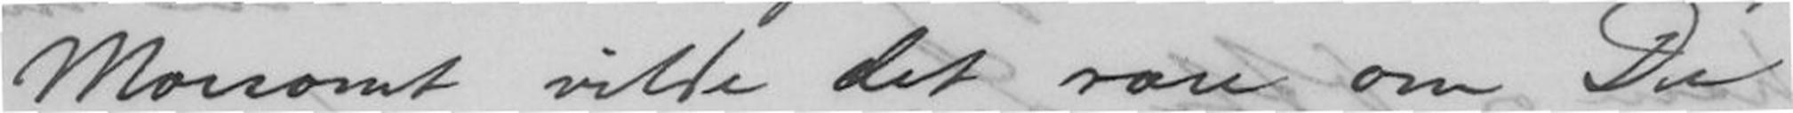Morsomt vilde det være om Du
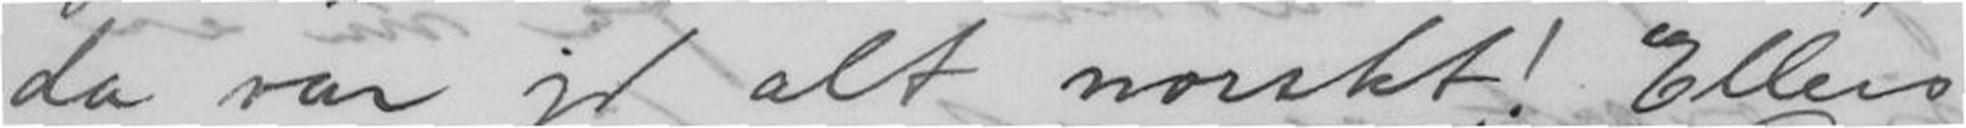da var jo alt norskt! Ellers
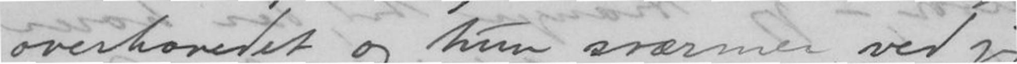overhovedet, og hun sværmer, ved jeg,
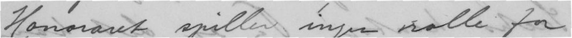Honoraret spiller ingen rolle for
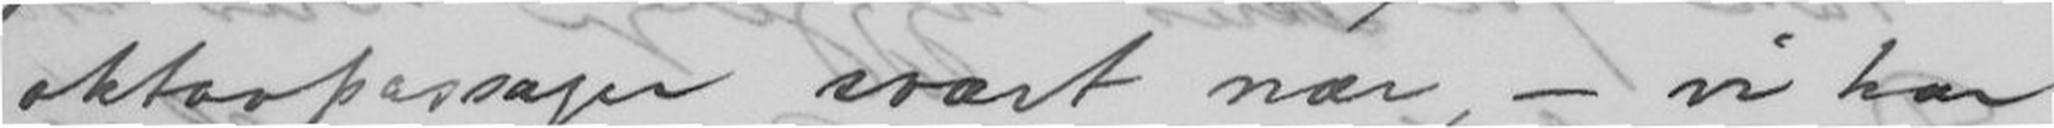oktavpassager svært nær, - vi har
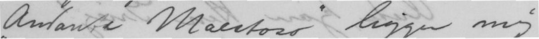"Andante Maestoso" ligger mig
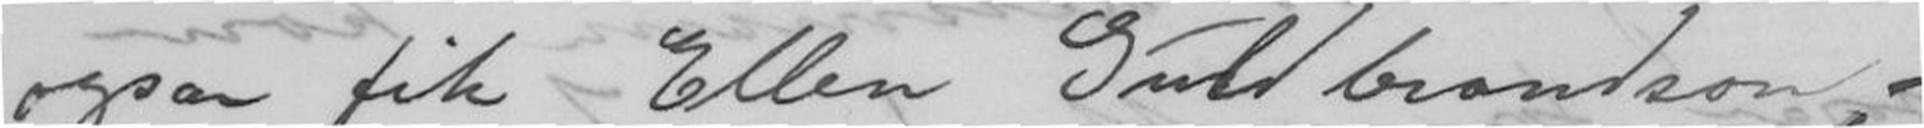ogsaa fik Ellen Guldbrandson,
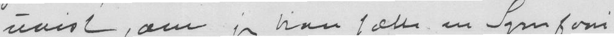uvist, om jeg kan sætte en Symfoni
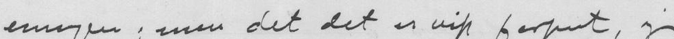eningen; men det det er vist forsent, jeg
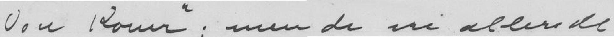"Vore Koner"; men de ere allerede
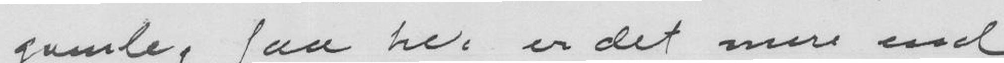gamle, saa her er det mere end
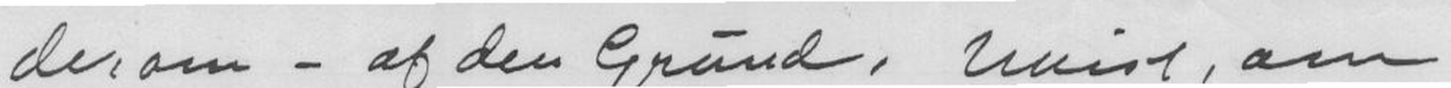derom - af den Grund, Uvist , om
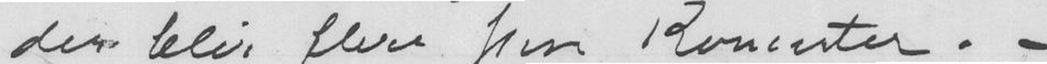der bliver flere store Koncerter.-
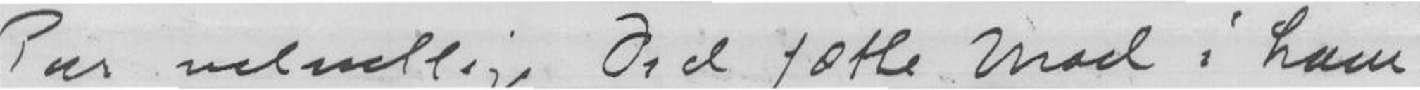Par velvillige Ord sætte mod i ham,
